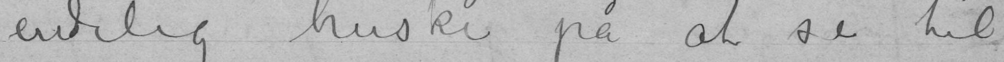endelig huske på at se til
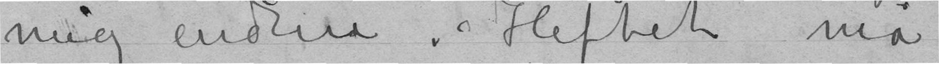mig endnu. Heftet må
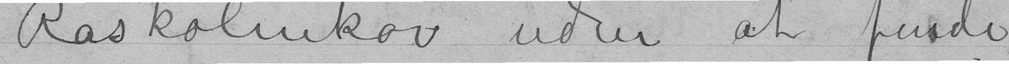Raskolnikov uden at finde
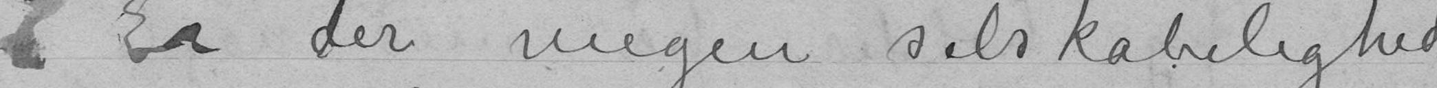Er der megen selskabelighed
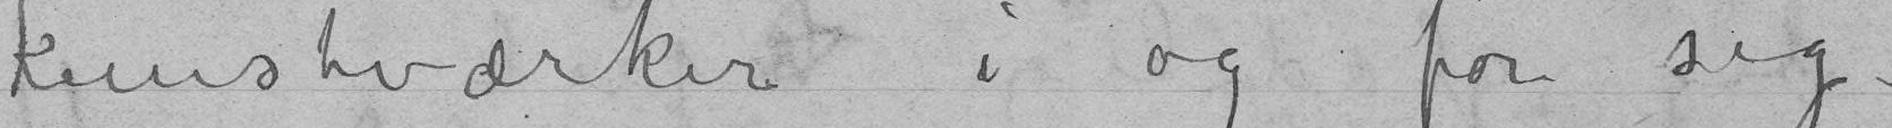kunstværker i og for sig.
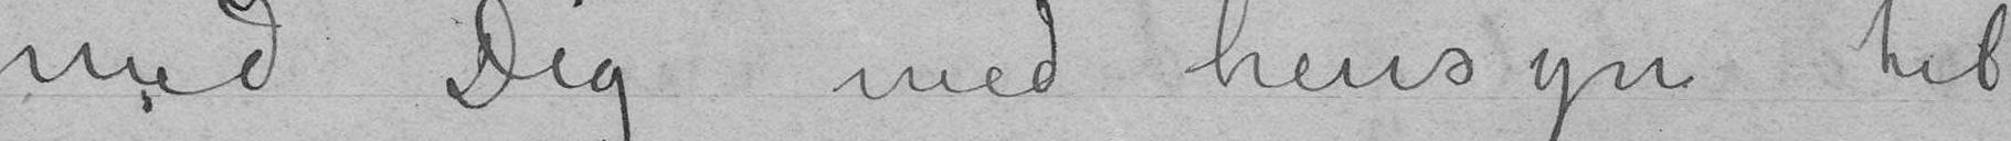med Dig med hensyn til
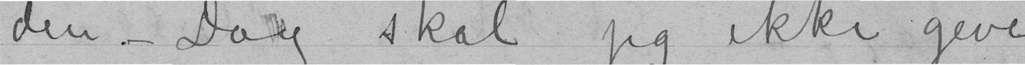den – Dog skal jeg ikke give
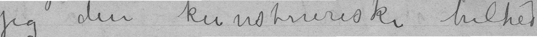jeg den kunstneriske helhed.
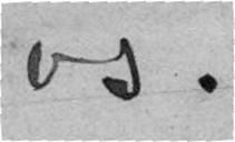os.
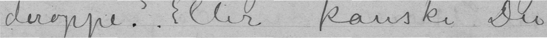deroppe? Eller kanske Du
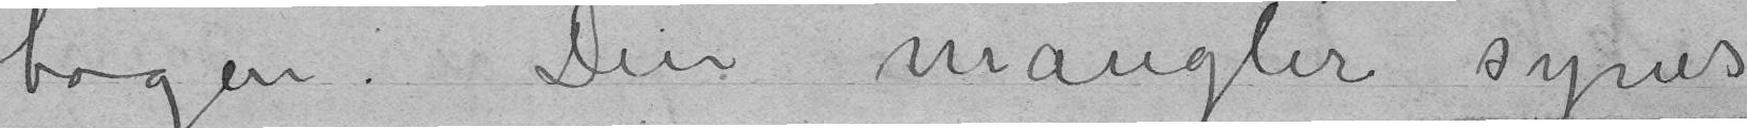bogen. Den mangler synes
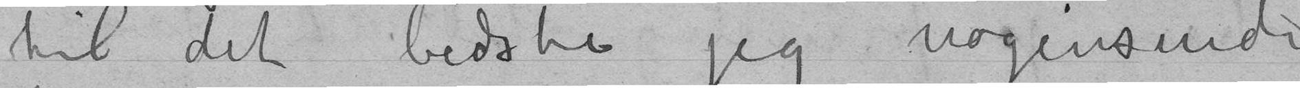til det bedste jeg nogensinde
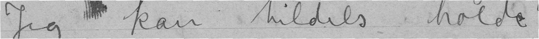Jeg kan tildels holde
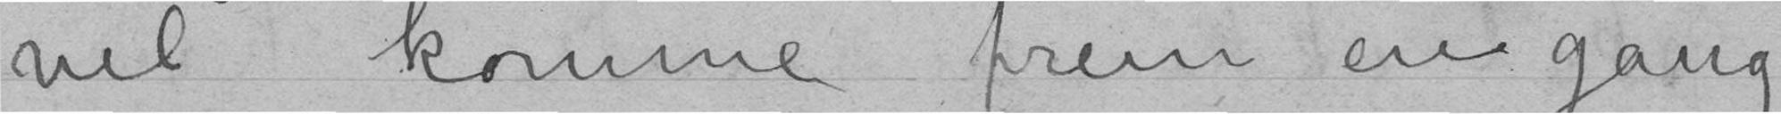vel komme frem engang
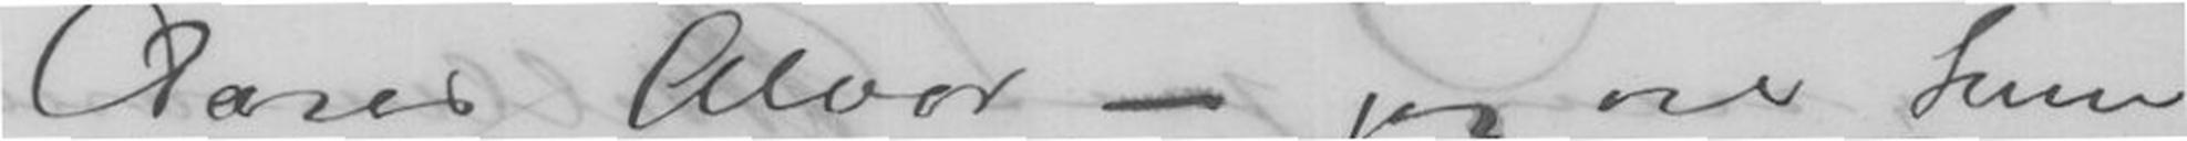Deres Alvor - jeg vil kun
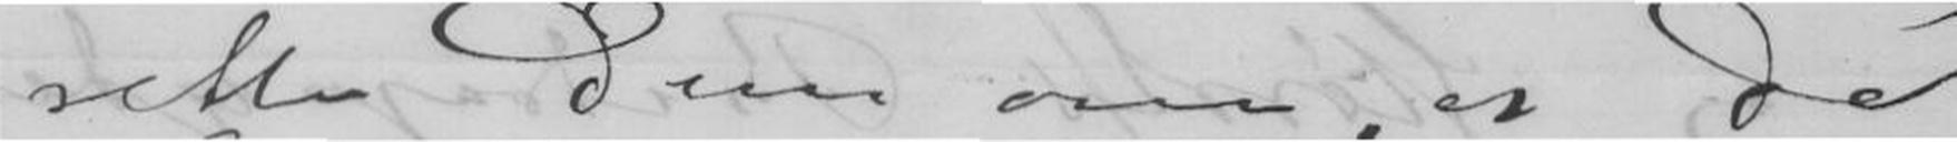rette Dem om at De
